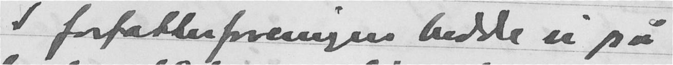I forfatterforeningen hadde vi på
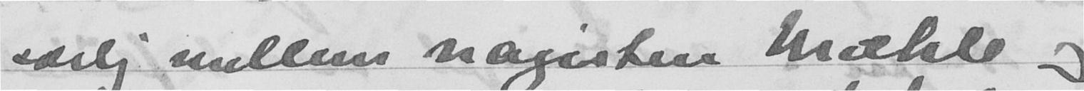særlig mellem nagisten Mæhle og
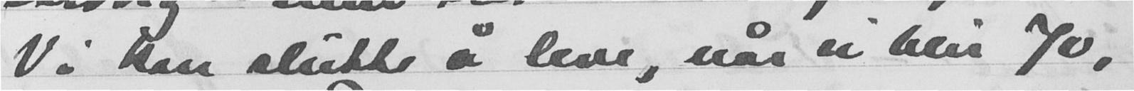Vi kan slutte å leve, når vi blir 70,
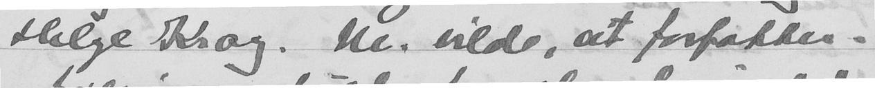Helge Krog. Vi vilde, at forfatter-
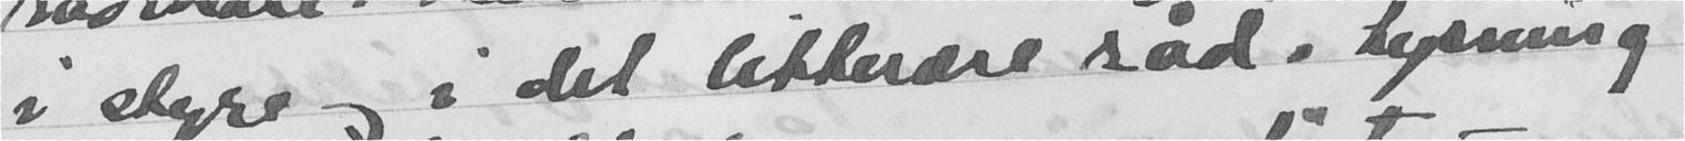i styre og i det litterære råd. Lysning
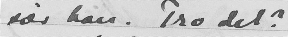sier han. Tro det?
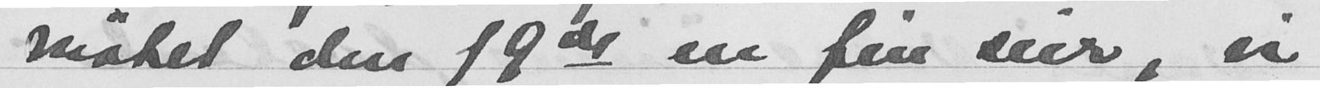møtet den 19de en fin seier, vi
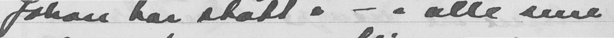Johan har stått i - i alle sine
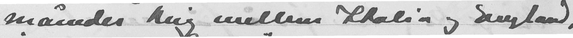måneder krig mellom Italia og England,
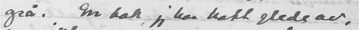også. En bok jeg har hatt glede av,
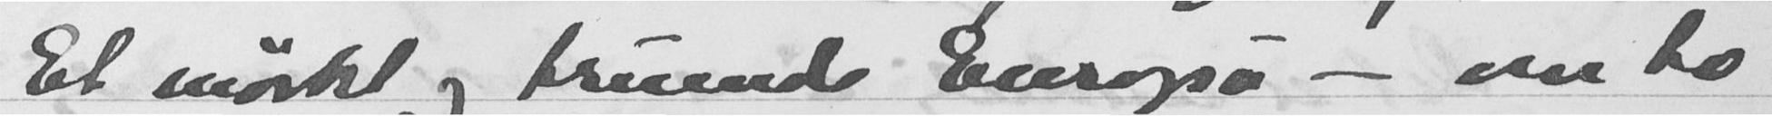Et mørkt og truende Europa - om to
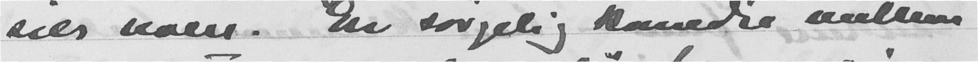sier noen. En sørgelig komedie mellom
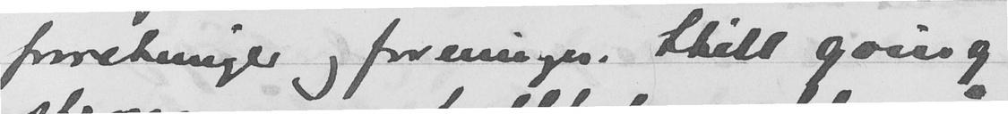forretninger og foreninger. Still going
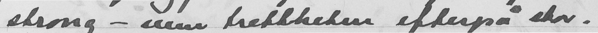strong - men trettheten efterpå stor.
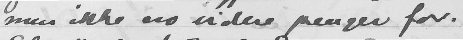men ikke noe videre penger for.
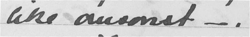like avsovnet -
